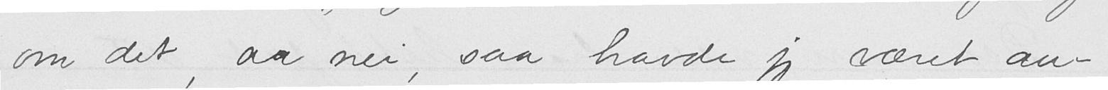om det, aa nei, saa havde jeg været au-
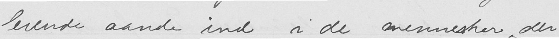levende aande ind i de mennesker, der
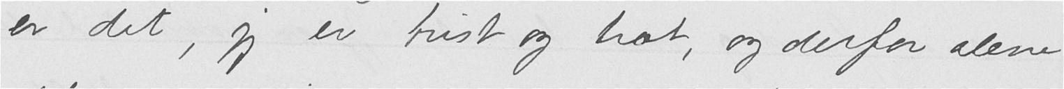er det, jeg er trist og træt, og derfor alene
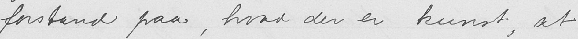forstand paa, hvad der er kunst, at
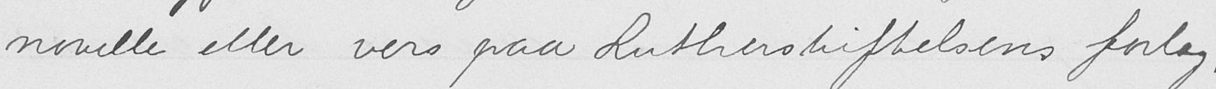novelle eller vers paa Lutherstiftelsens forlag,
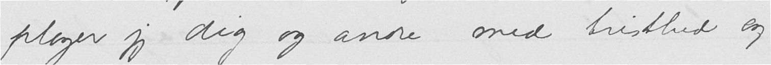plager jeg dig og andre med tristhed og
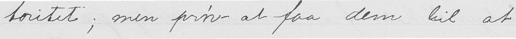toritet; men prøv at faa dem til at
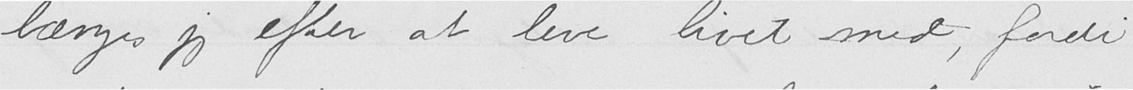længes jeg efter at leve livet med, fordi
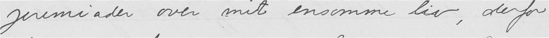jeremiader over mit ensomme liv, derfor
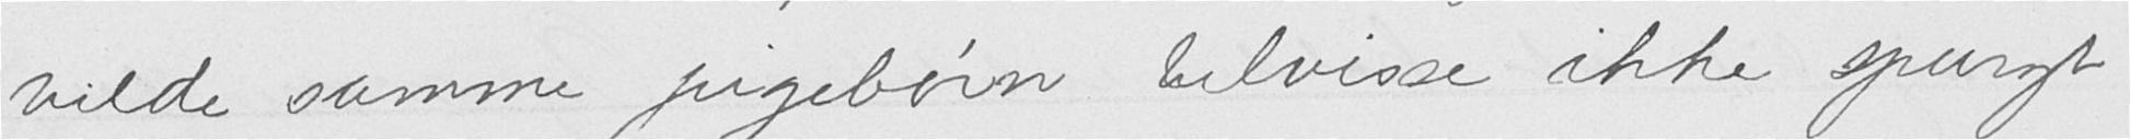vilde samme pigebørn tilvisse ikke spurgt
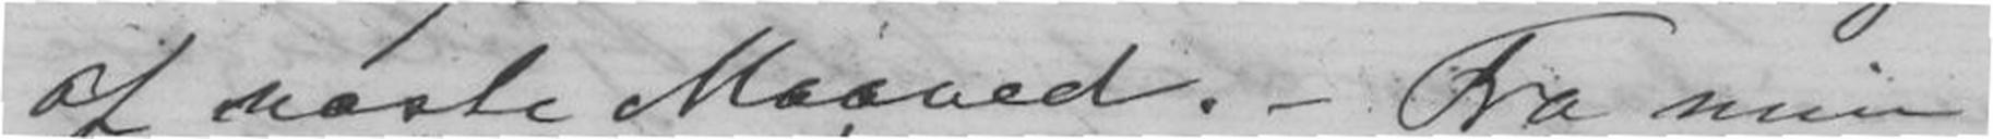af næste Maaned. - Fra min
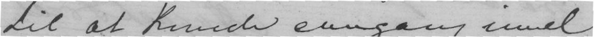til at kunde enngang imel
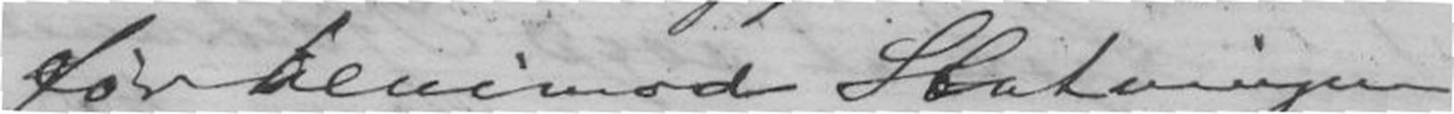før henimod Slutningen
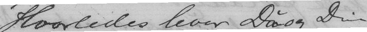Hvorledes lever Du og Din
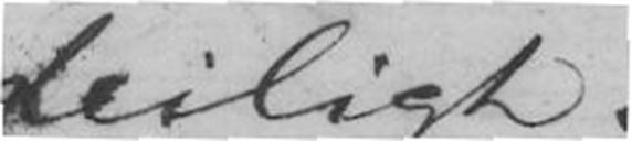deiligt.
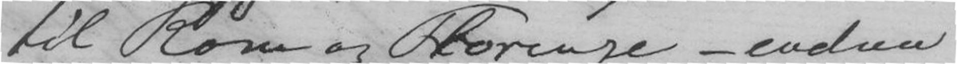til Rom og Florenze - endnu
